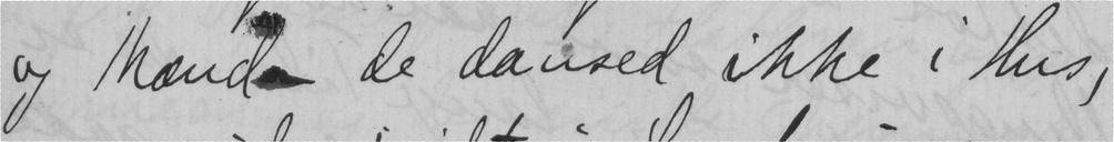og Mænd. de dansed ikke i Hus,
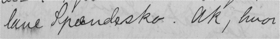lave spændesko. Ak, hvor
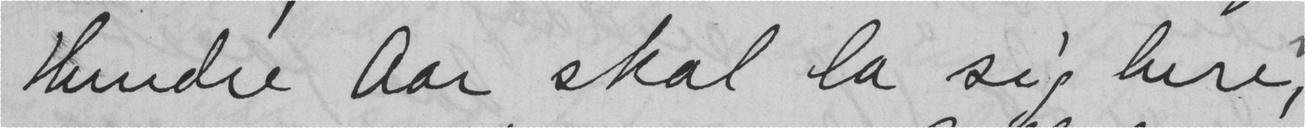Hundre aar skal la sig lure,
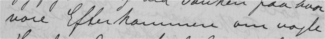vore Efterkommere om nogle
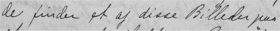de finder et af disse Billeder paa
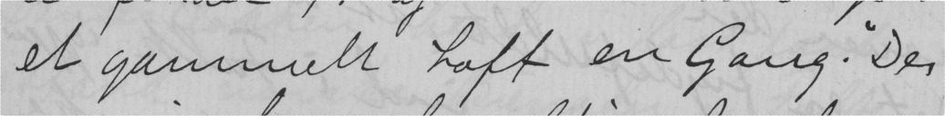et gammelt Loft en Gang. "Der
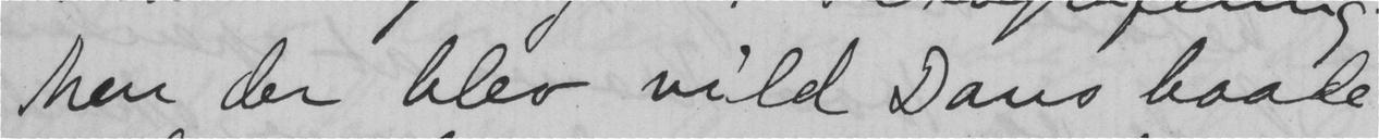Men der blev vild Dans baade
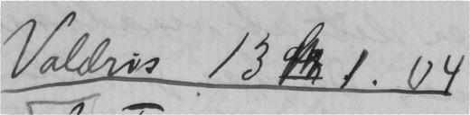Valdris 13  .1.04
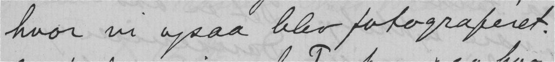hvor vi ogsaa blev fotograferet.
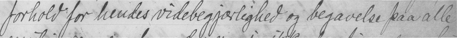forhold for hendes videbegjærlighed og begavelse paa alle
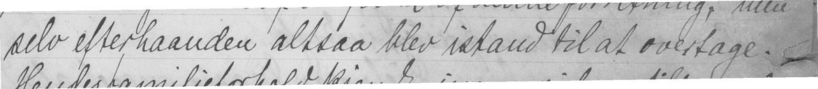selv efterhaanden altsaa blev istand til at overtage.
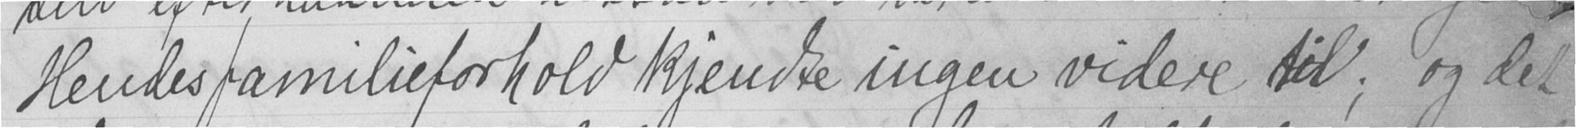Hendes familieforhold kjendte ingen videre til; og det
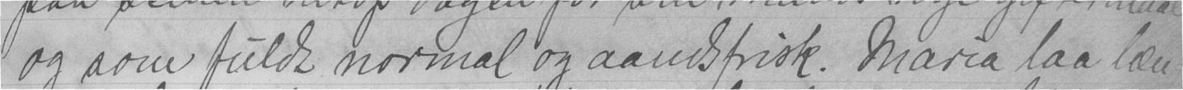og som fuldt normal og aandsfrisk. Maria laa læn-
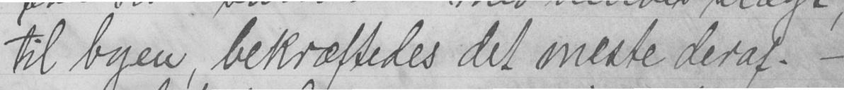til byen, bekræftedes det meste deraf. -
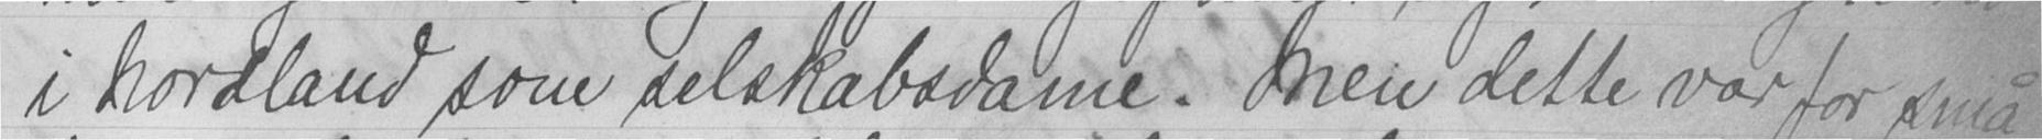i Nordland som selskabsdame. Men dette var for små
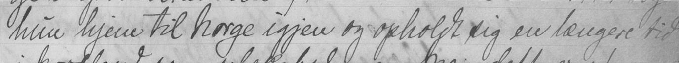hun hjem til Norge igjen og opholdt sig en længere tid
